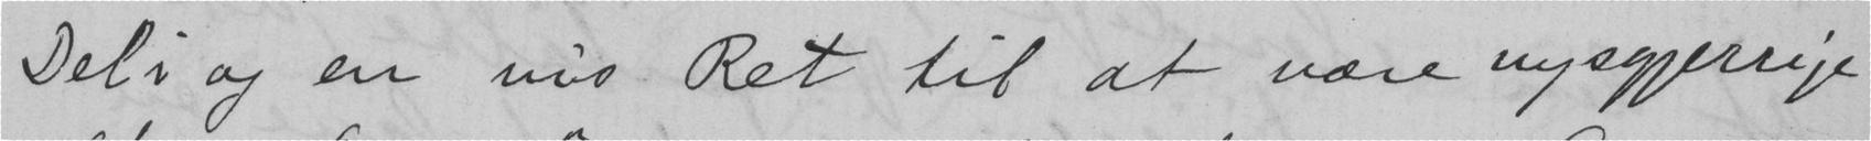Del i og en vis Ret til at være nyegjerrige
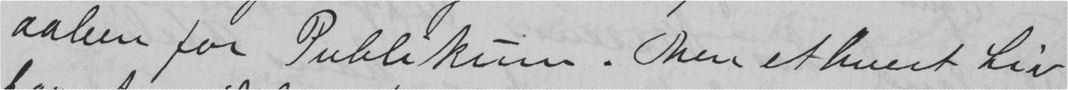aaben for Publikum. Men ethvert Liv
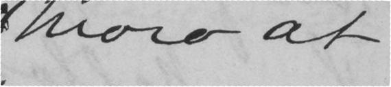moro at
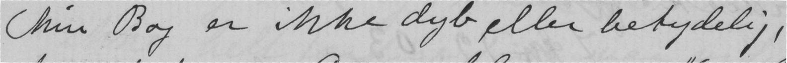Min Bog er ikke dyb eller betydelig,
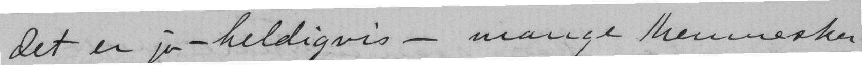det er jo - heldigvis - mange mennesker
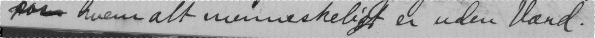for hvem alt menneskeligt er uden Værd.
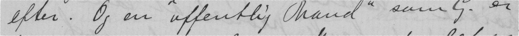efter. Og en "offentlig Mand" som G. er
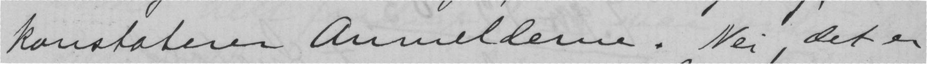konstaterer anmelderne. Nei, det er
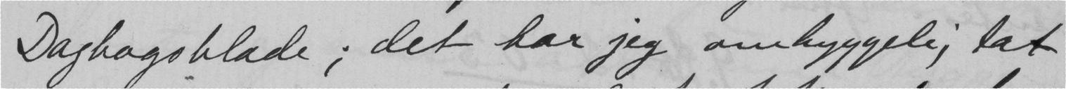Dagbogsblade; det har jeg omhyggelig lat
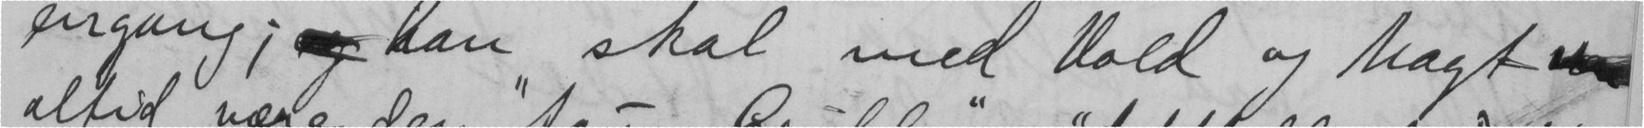engang;  han skal med Vold og Magt
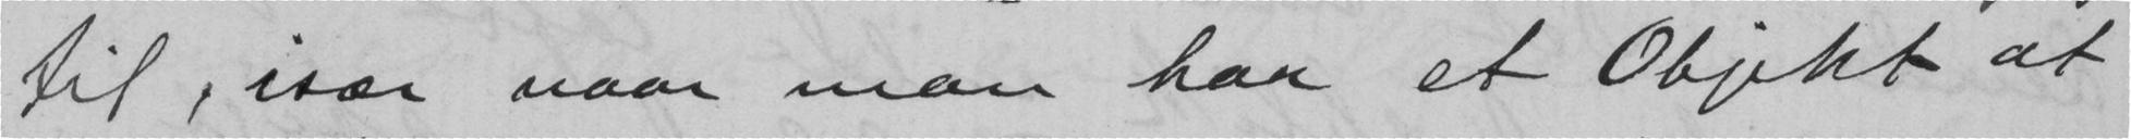til, især naar man har et Objekt at
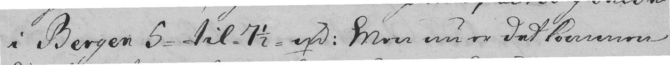i Bergen 5 til 7 ½ mk. Men nu er det kommen
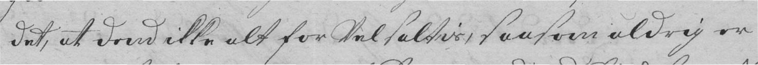det, at dend ikke alt for Vel saltis, saasom aldrig er
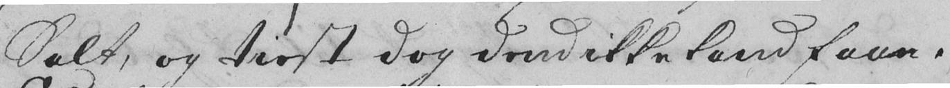Salt, og tiest dog dend ikke kand faae.
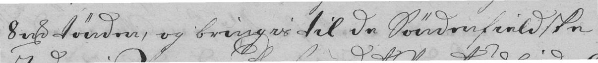8 mk. tønden, og bringis til de Søndenfieldske
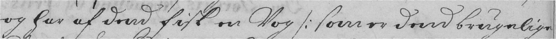og har af dend Fisk en Vog /: som er dend brugelige
